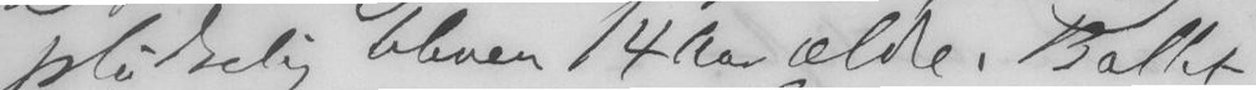pludselig bleven 14 Aar ældre. Ballet
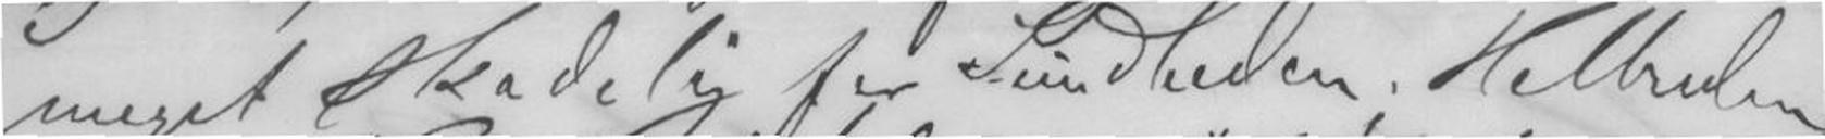meget skadelig for Sundheden. Helbreden
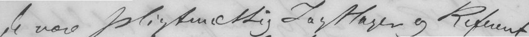de være pligtmæssig Iagtager og Referent
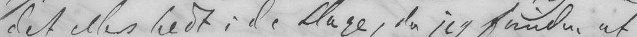det ellers hedt i de Dage, da jeg foruden at
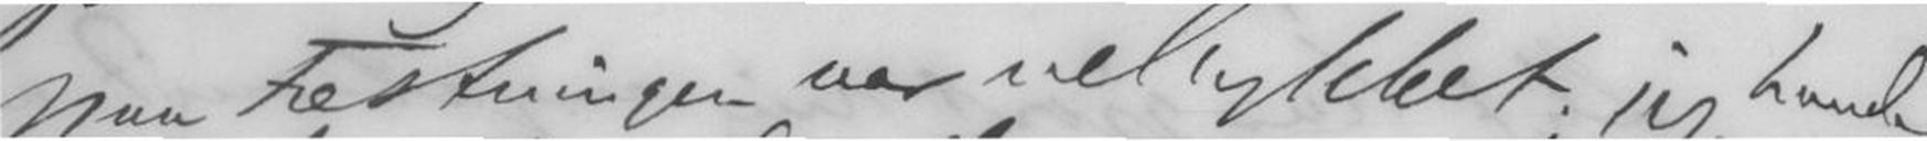paa Festningen var vellykket; jeg havde
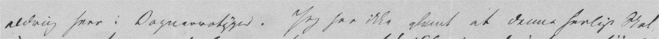aldrig seer i Daguerrotyper. Jeg har ikke glemt at denne herlige Skat
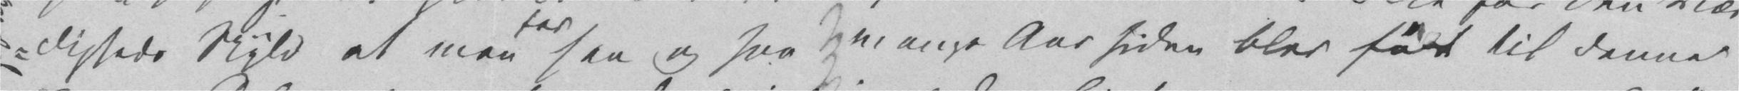digheds Skyld at man for saa og saa mange Aar siden blev født til denne
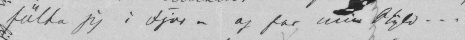følte jeg i Fjor – og for min Skyld – –.
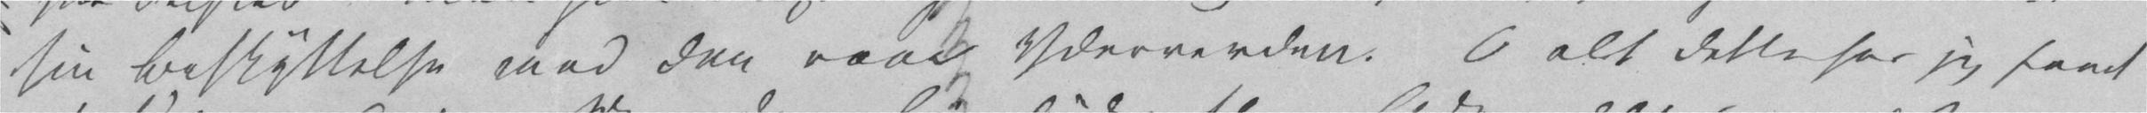sin Beskyttelse mod den raae Yderverden. O alt dette har jeg faaet
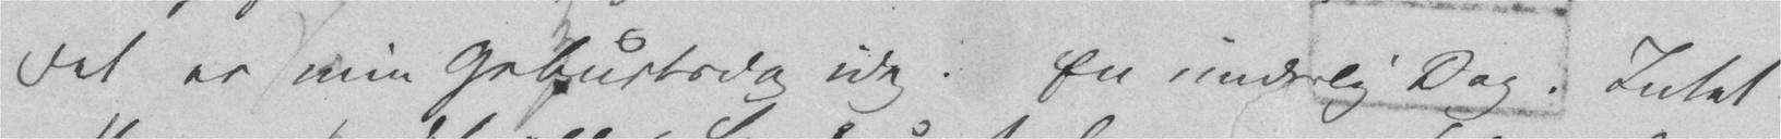Det er min Geburtsdag idag. En underlig Dag. Intet
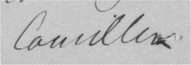Camilla
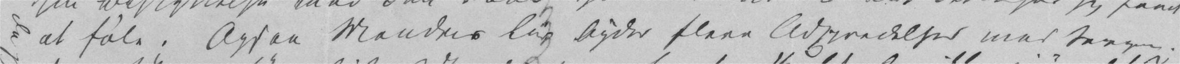at føle. Ogsaa Mandens Liv byder flere Adspredelser mod Sorgen.
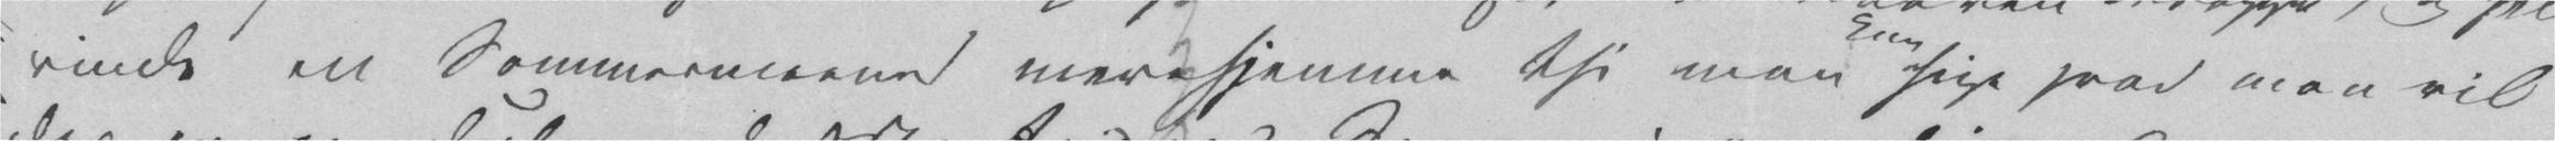vinde en Sommermaaned mere hjemme thi man kan sige hvad man vil
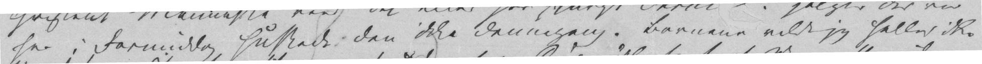her i Formiddag huskede den ikke dennegang. Børnene vilde jeg heller ikke
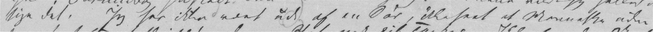sige det. Jeg har ikke været ude af en Dør, ikke seet et Menneske uden
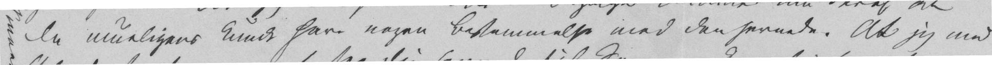Du mueligens kunde have nogen Bestemmelse med den hernede. At jeg med
